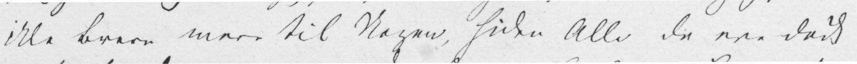ikke Breve mere til Nogen, siden Alle de ere døde
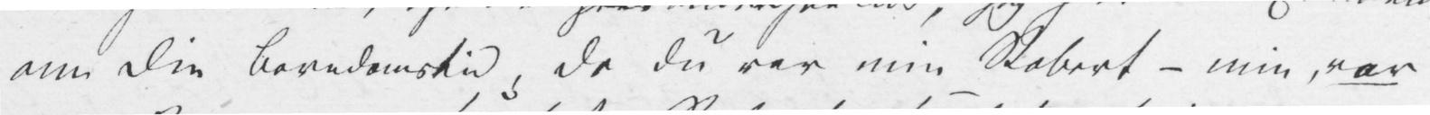om Din Barndomstid, da Du var min Robert – min, vor
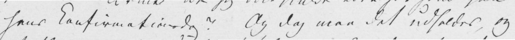hans Confirmationsdag? Og dog maa det udholdes, og
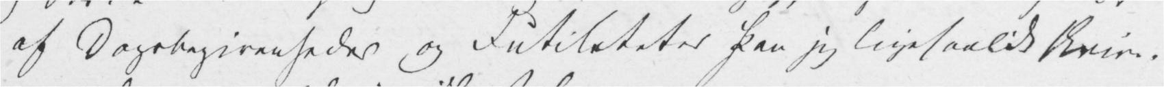af Dagsbegivenheder og Futiliteter kan jeg ligesaalidt skrive.
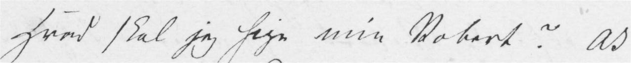Hvad skal jeg sige min Robert? Ak
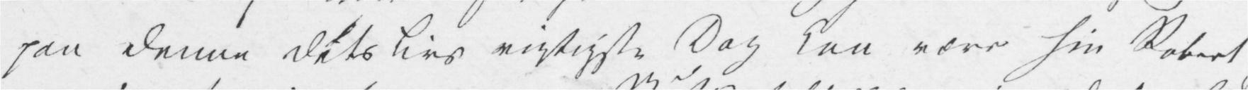paa denne dets Livs vigtigste Dag kan være sin Robert
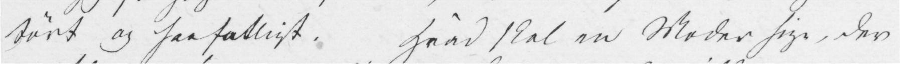tørt og saa fattigt. Hvad skal en Moder sige, der
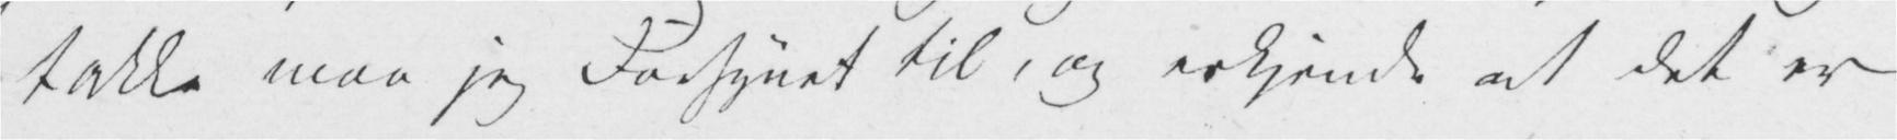takke maa jeg Forsynet til, og erkjende at det er
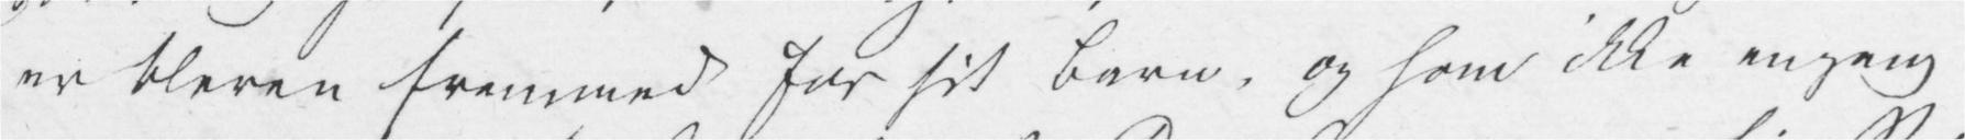er bleven fremmed for sit Barn, og som ikke engang
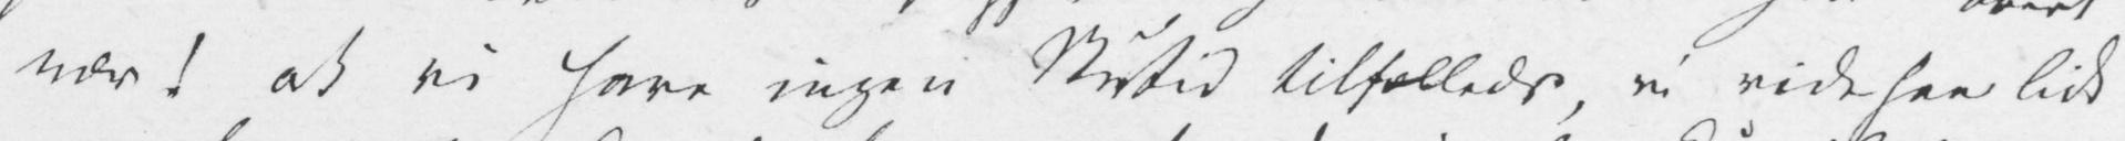nær! ak vi have ingen Nutid tilfælleds, vi vide saa lidt
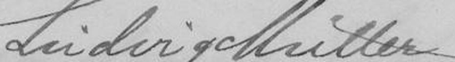Ludvig Müller.
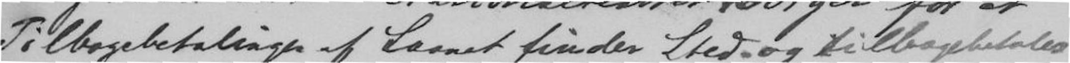Tilbagebetalingen af Laanet finder Sted, og til bagebetales
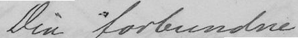Din forbundne
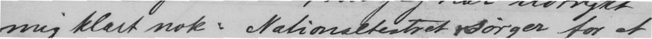mig klart nok: Nationalteatret sørger for at
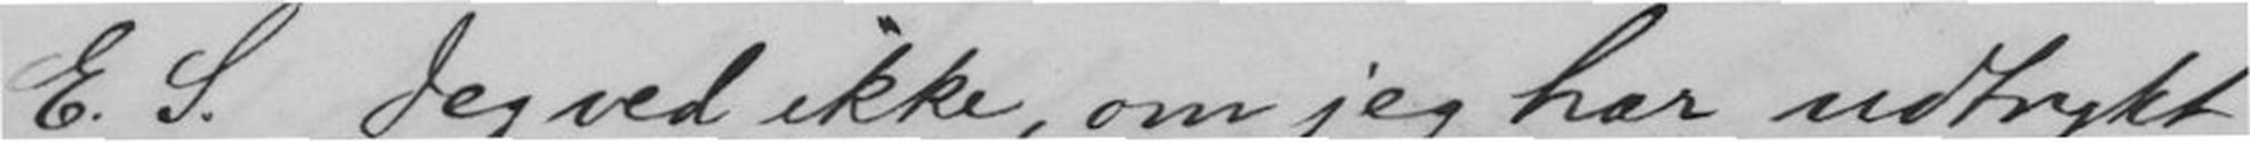E. S. Jeg ved ikke, om jeg har udtrykt
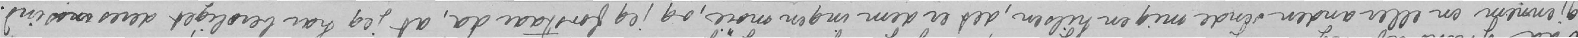gjennem en eller anden sende mig en hilsen, det er dem ingen møie, og jeg forstaar da, at jeg har beroliget deres insind.
